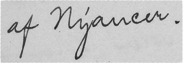af Nyancer.
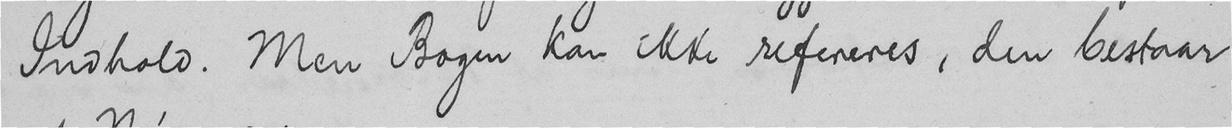Indhold. Men Bogen kan ikke refereres, den bestaar
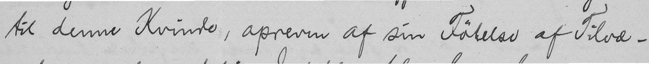til denne Kvinde, opreven af sin Følelse af Tilvæ-
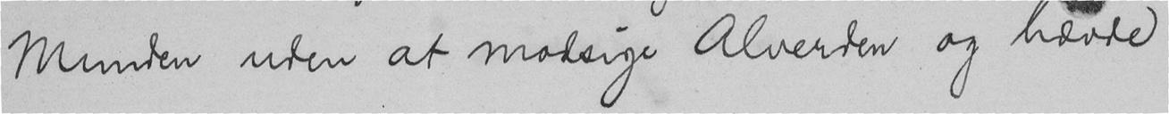Munden uden at modsige Alverden og hævde
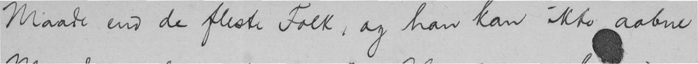Maade end de fleste Folk, og han kan ikke aabne
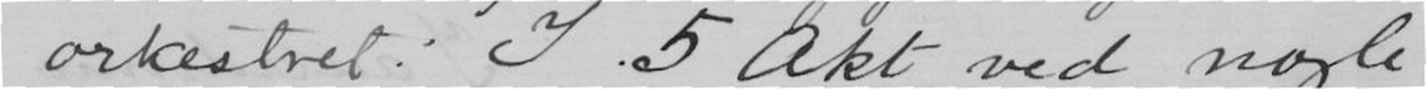orkestret. I 5 Akt ved nogle
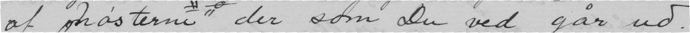af Nøsterne der som Du ved går ud.
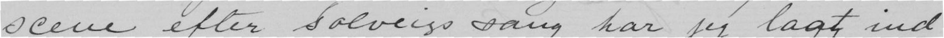scene efter Solveigs sang har jeg lagt ind
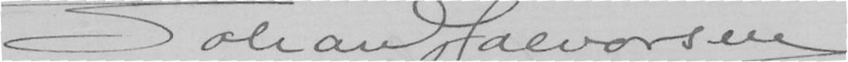Johan Halvorsen
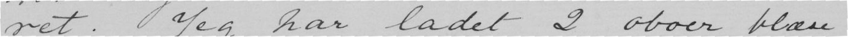ret. Jeg har ladet 2 oboer blæse
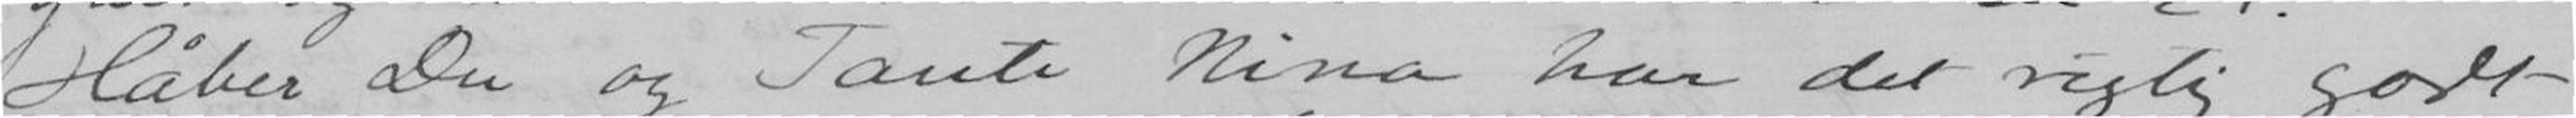Håber Du og Tante Nina har det rigtig godt
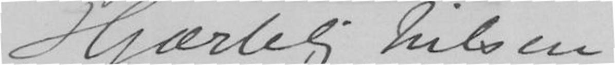Hjærtelig hilsen
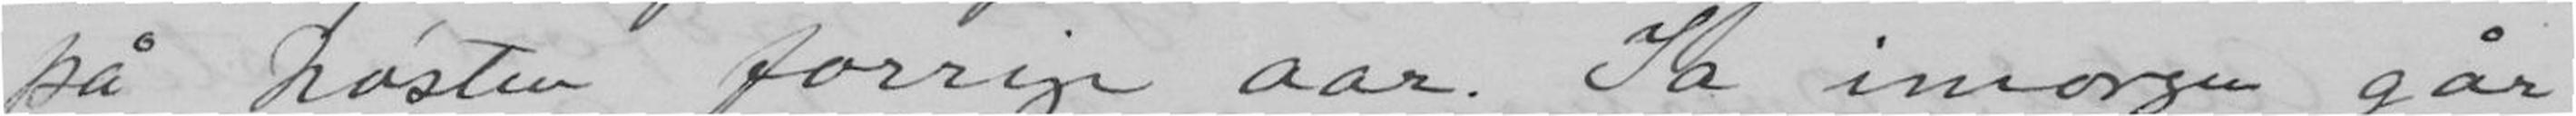på høsten forrige aar. Ja imorgen går
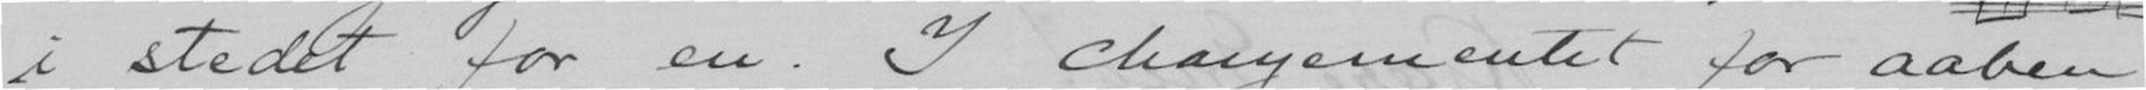i stedet for en. I changementet for aaben
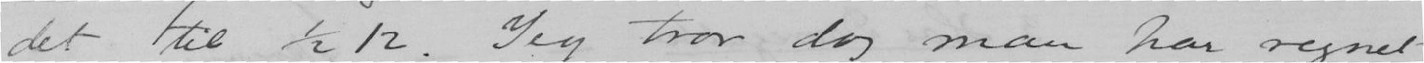det til ½12. Jeg tror dog man har regnet
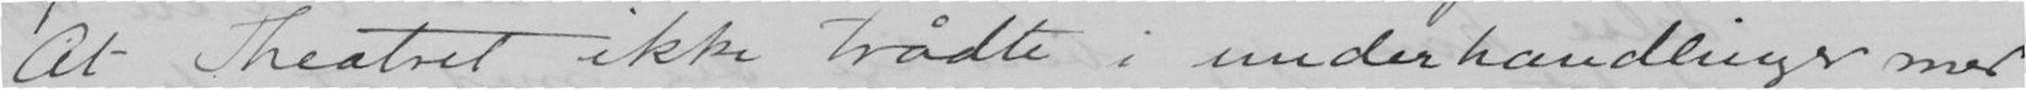At Theatret ikke trådte i underhandlinger med
